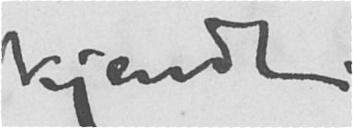kjendt.
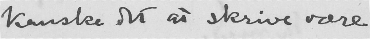kanske det at skrive være
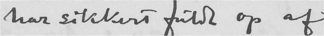har sikkert fuldt op af
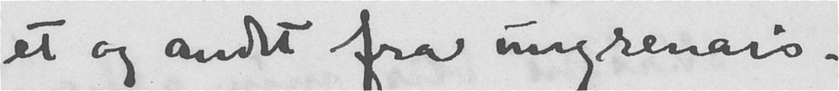et og andet fra ungrenais-
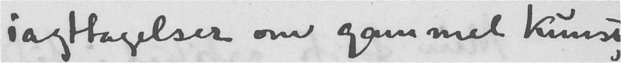lagttagelser om gammel kunst,
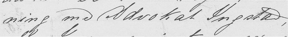ning med Advokat Ingstad,
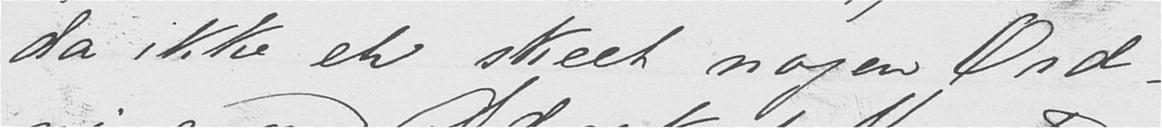da ikke er skeet nogen Ord-
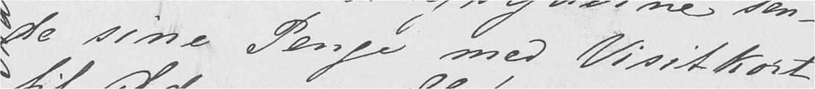de sine Penge med Visitkort,
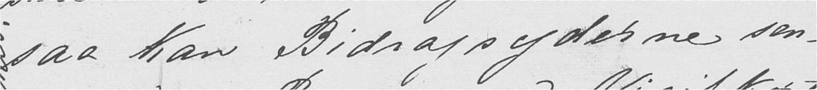saa kan Bidragsyderne sen-
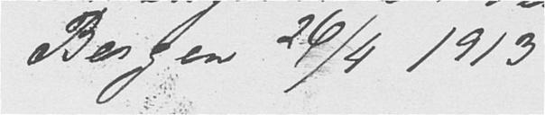Bergen 26/4 1913
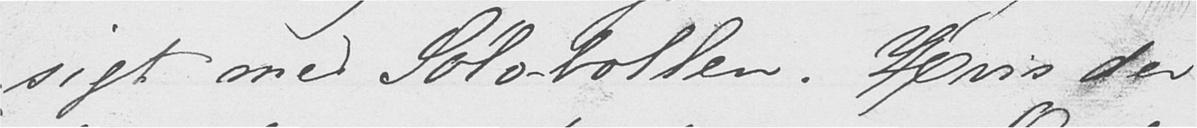sigt med Sølv-bollen. Hvis der
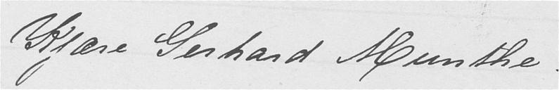Kjære Gerhard Munthe.
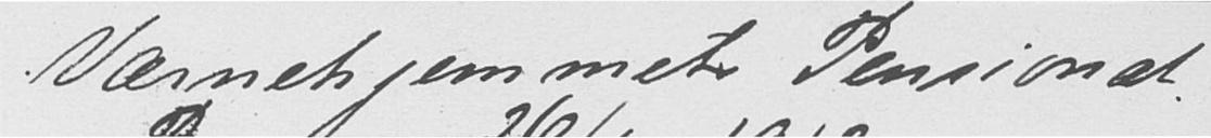Værnehjemmet Pensionat
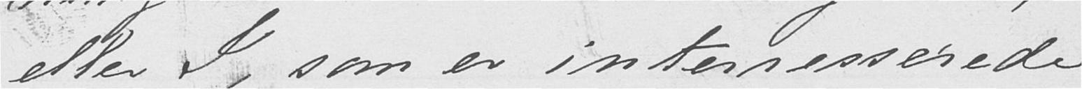eller I, som er interesserede
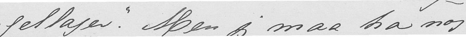gellager. Men jeg maa ha nog
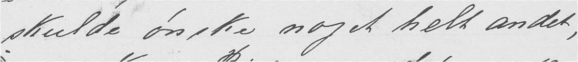skulde ønske noget helt andet,
